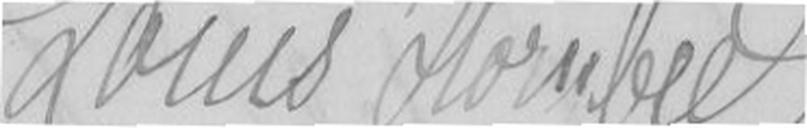Louis Hornbeck
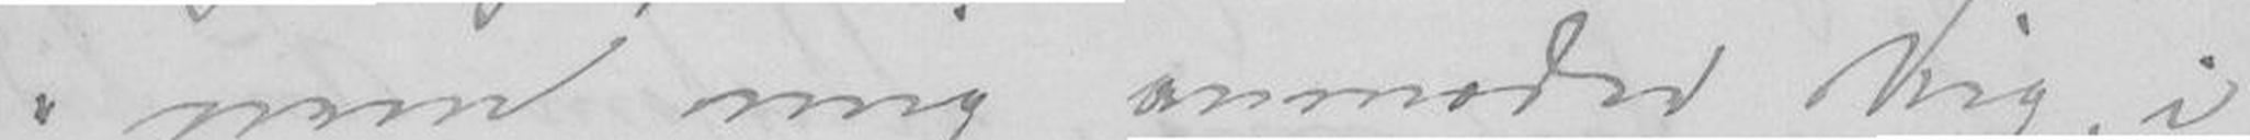nem mig anmoder Dig, i
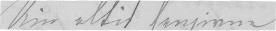Din altid hengivne
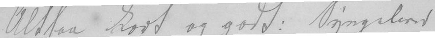Altsaa kort og godt: Syngelærer
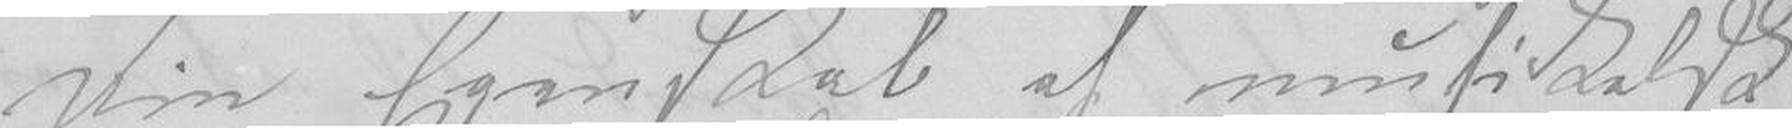Din Egenskab af musikalsk
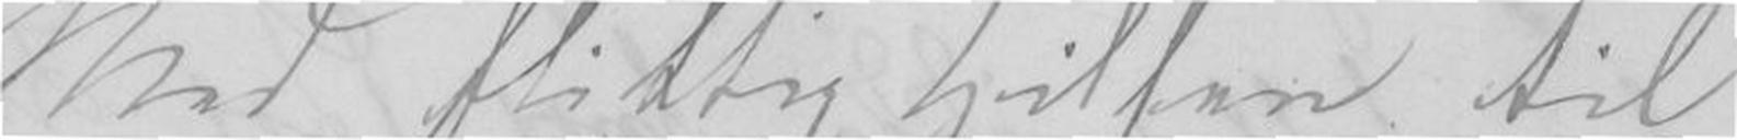Med flittig hilsen til
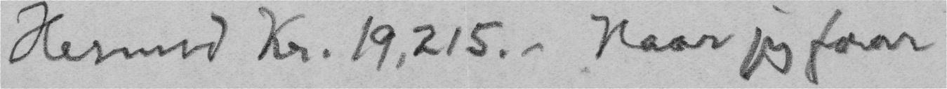Hermed Kr. 19,215. - Naar jeg faar
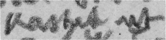kastet ut
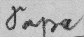Papa
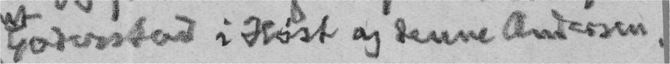Goderstad i Høst og denne Andersen.
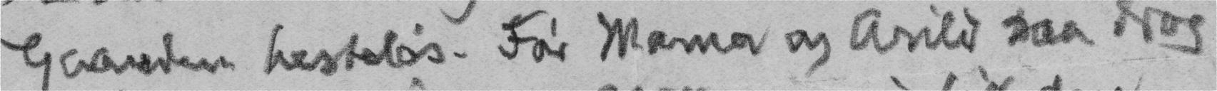Gaarden hesteløs. Før Mama og Arild saa drog
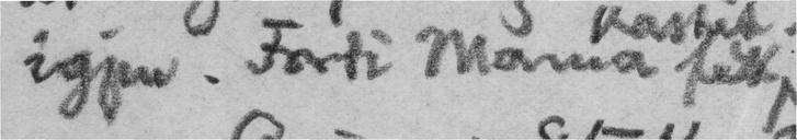igjen. Fordi Mama fik
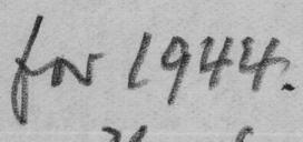for 1944.
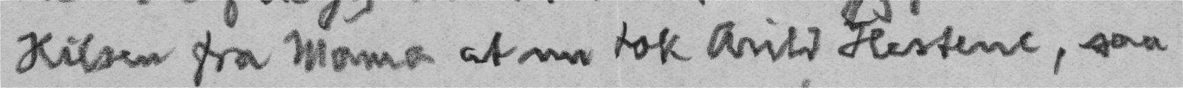Hilsen fra Mama at nu tok Arild Hestene, saa
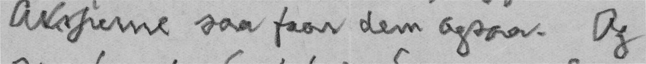Aksjerne saa faar dem ogsaa. Og
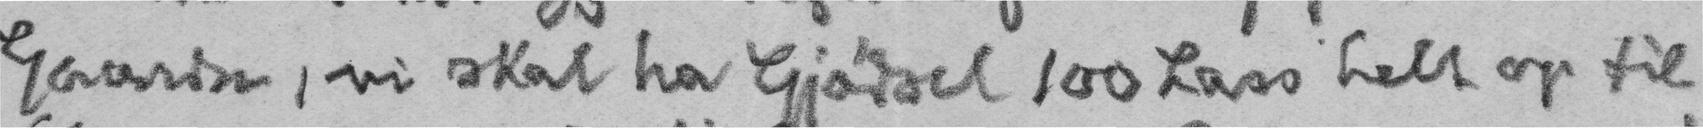Gaarden, vi skal ha Gjødsel 100 Lass helt op til
